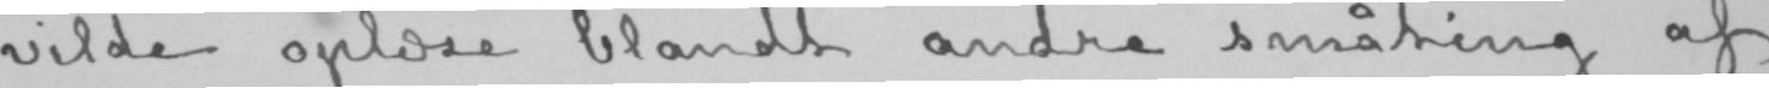vilde oplæse blandt andre småting af
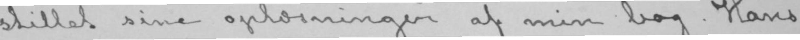stillet sine oplæsninger af min bog. Hans
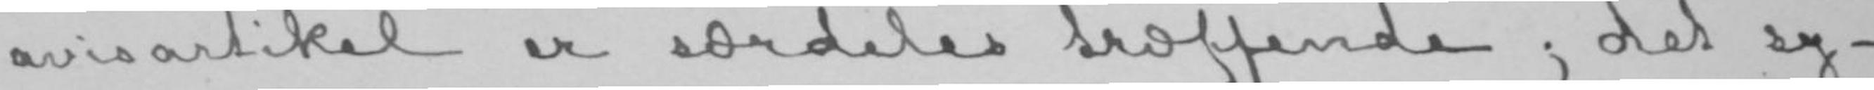avisartikel er særdeles træffende; det sy-
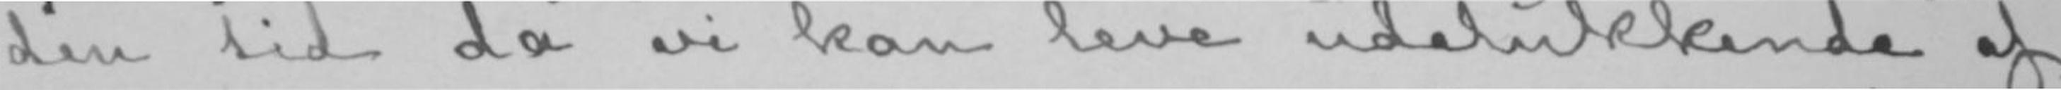den tid da vi kan leve udelukkende af
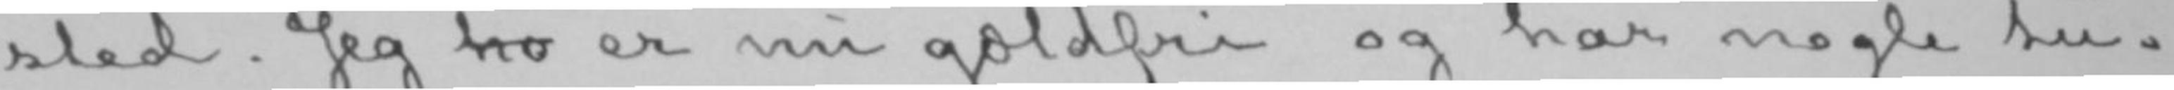sted. Jeg h er nu gældfri og har nogle tu-
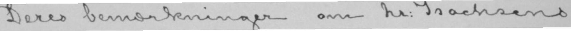Deres bemærkninger om hr: Isachsens
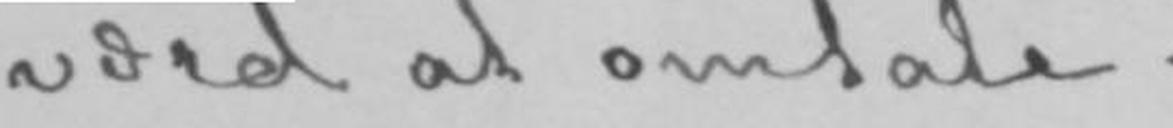værd at omtale.
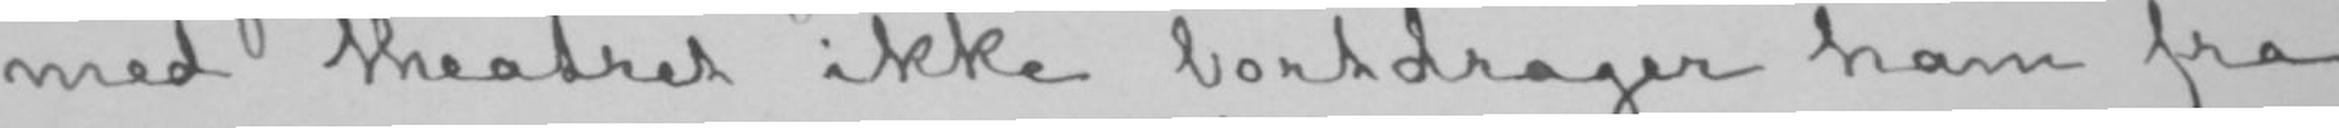med theatret ikke bortdrager ham fra
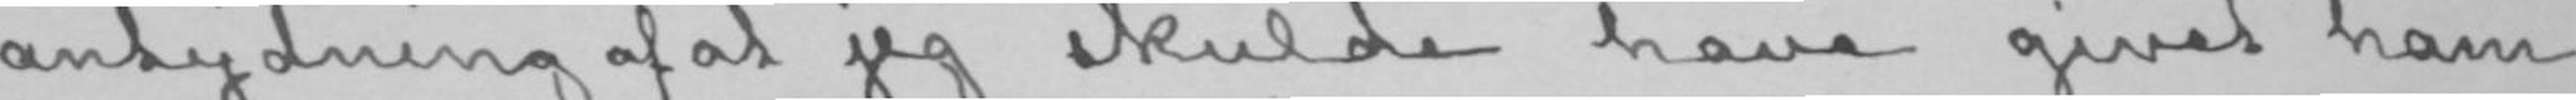antydning af at jeg skulde have givet ham
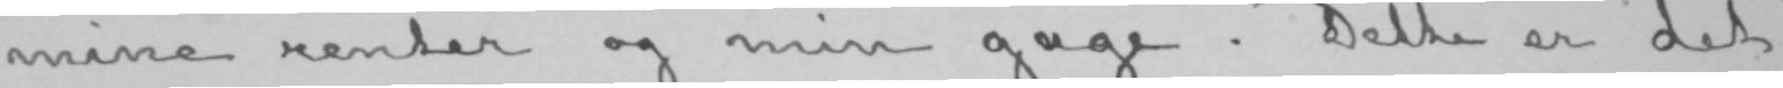mine renter og min gage. Dette er det
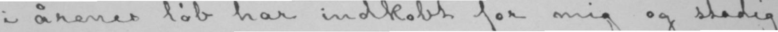i årenes løb har indkøbt for mig og stadig
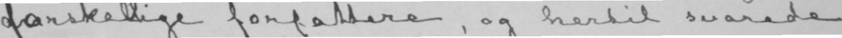anforskellige forfattere, og hertil svarede
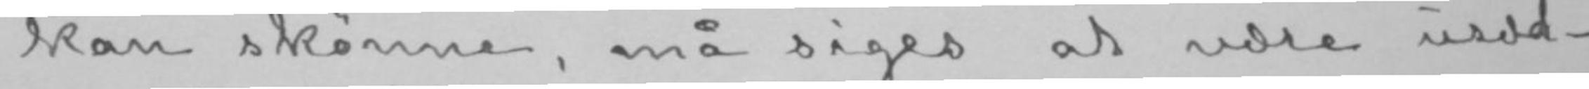jeg kan skønne, må siges at være usæd-
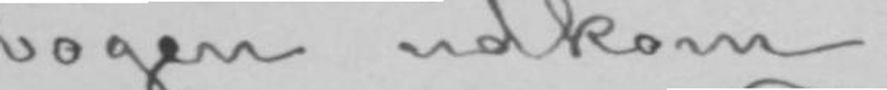bogen udkom.
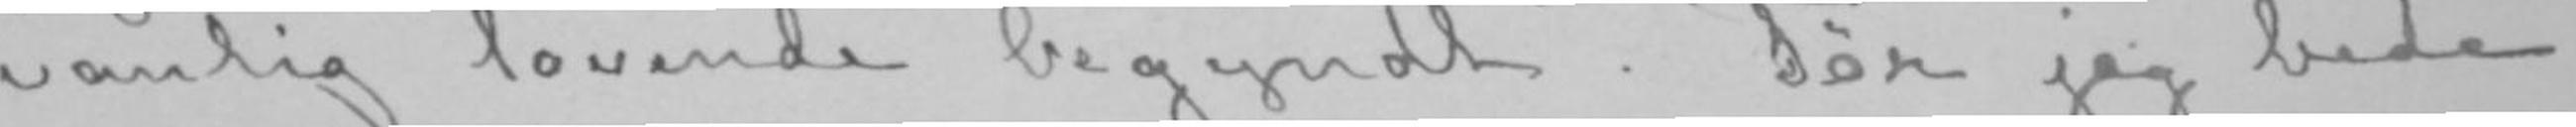vanlig lovende begyndt. Tør jeg bede
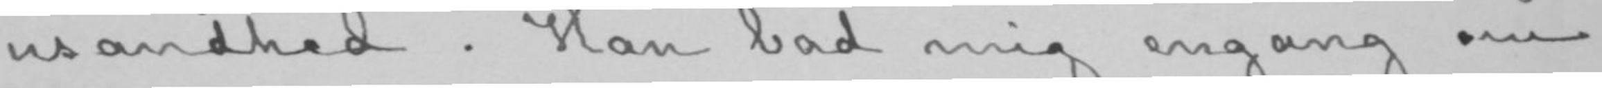usandhed. Han bad mig engang om
